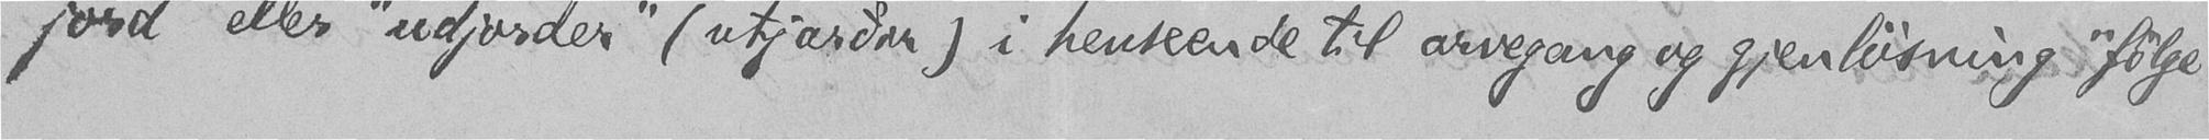jord" eller "udjorder" (utjarðar) i henseende til arvegang og gjenløsning "følge
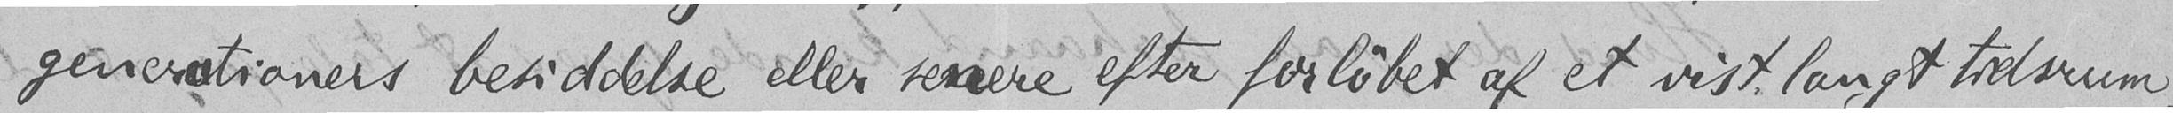generationers besiddelse eller senere efter forløbet af et vist langt tidsrum.
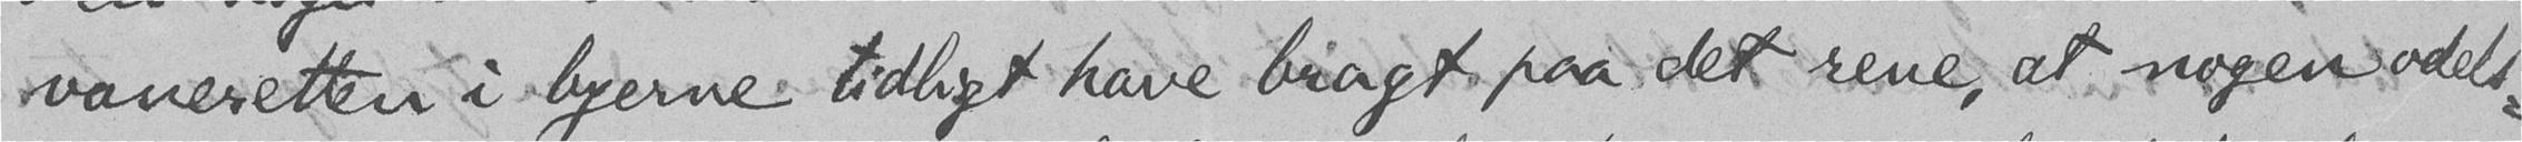vaneretten i byerne tidligt have bragt paa det rene, at nogen odels-
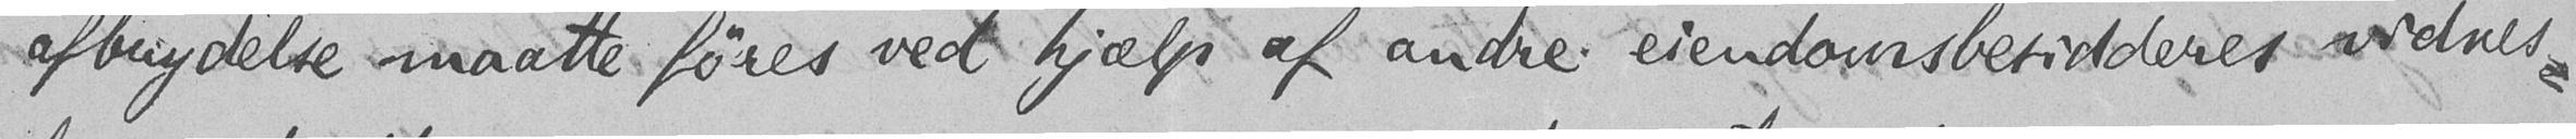afbrydelse maatte føres ved hjælp af andre eiendomsbesidderes vidnes-
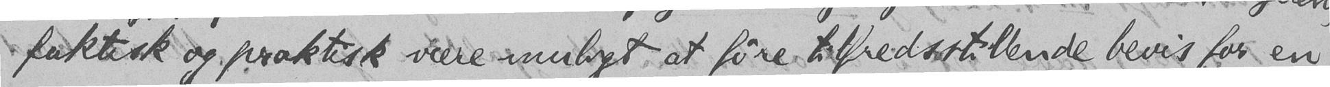faktisk og praktisk være muligt at føre tilfredsstillende bevis for en
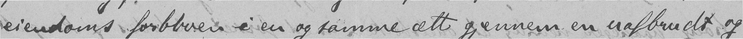eiendoms forbliven i en og samme ætt gjennem en uafbrudt og
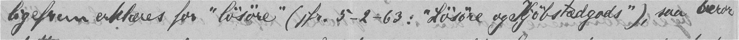ligefrem erklæres for "løsøre" (jfr. 5-2-63: "Løsøre og Kjøbstædgods"), saa beror
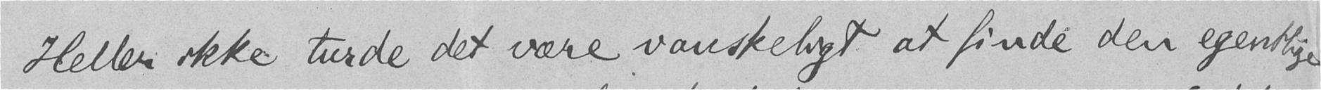Heller ikke turde det være vanskeligt at finde den egentlige
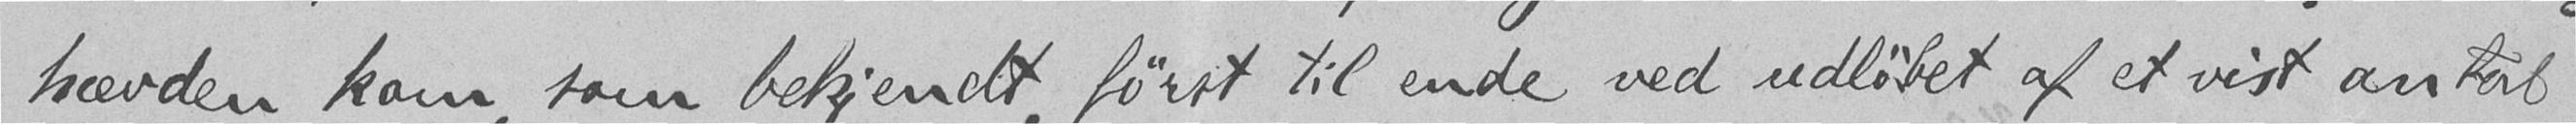hævden kom, som bekjendt, først til ende ved udløbet af et vist antal
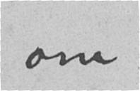om.
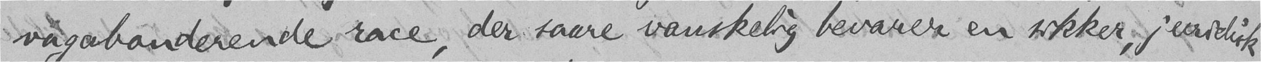vagabonderende race, der saare vanskelig bevarer en sikker, juridisk
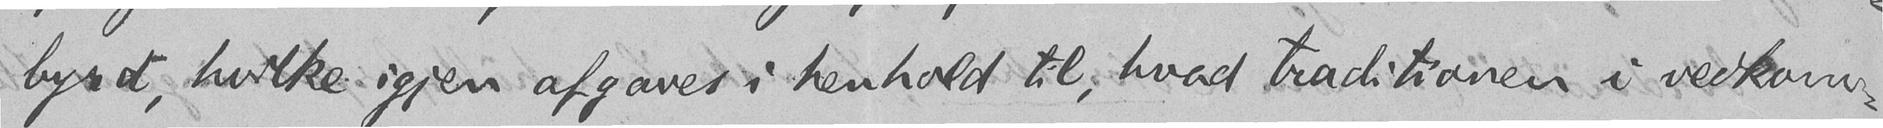byrd, hvilke igjen afgaves i henhold til, hvad traditionen i vedkom-
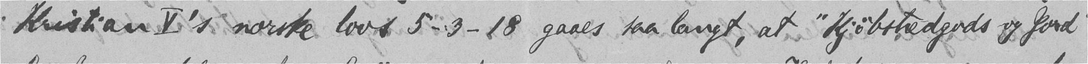Kristian V's norske lovs 5-3-18 gaaes saa langt, at "Kjøbstædgods og Jord"
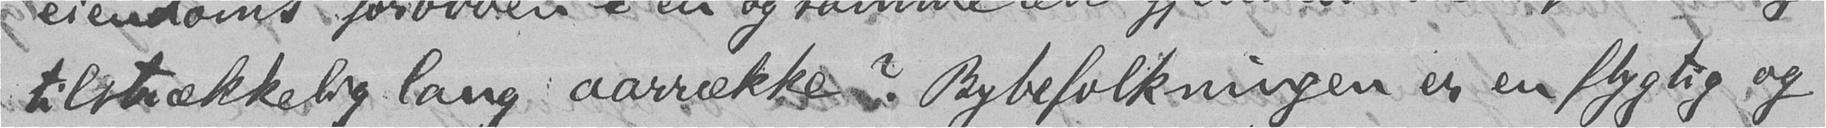tilstrækkelig lang aarrække? Bybefolkningen er en flygtig og
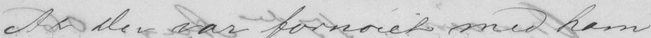At Du var fornøiet med ham
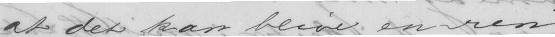at det kan blive en ren
